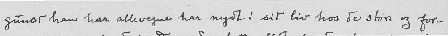gunst han har allevegne har nydt i sit liv hos de store og for-
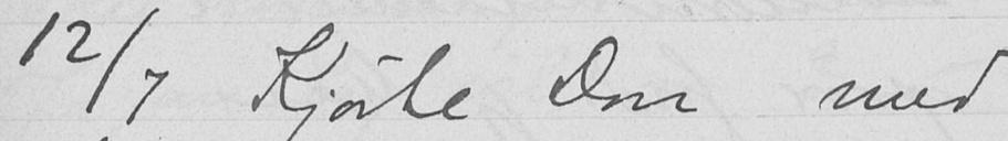12/7 Kjørte Don med
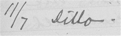11/7 Ditto.
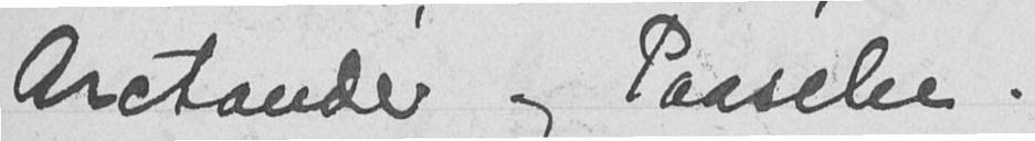Arctander og Paasche.
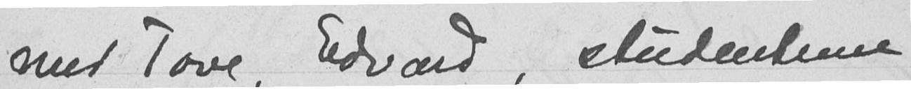med Tove, Edvard, studenterne
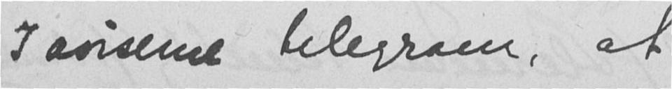I aviserne telegram, at
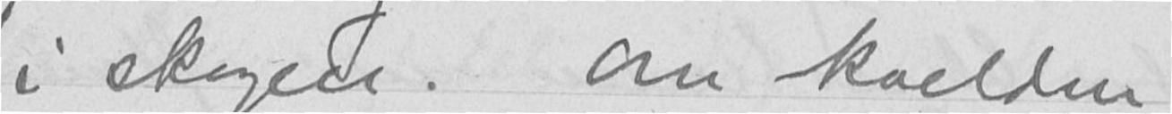i skogen. Om kvelden
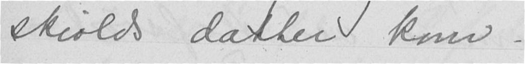skiolds datter kom.
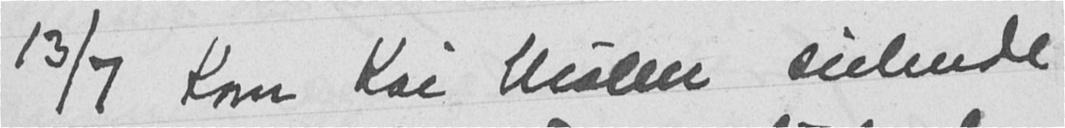13/7 Kom Kai Møller seilende
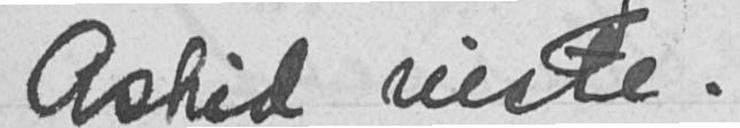Astrid reiste.
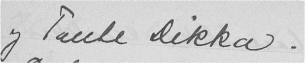og Tante Dikka.
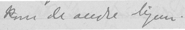kom de andre hjem.
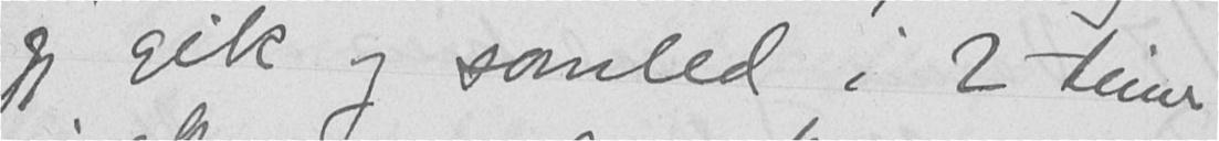jeg gik og somled i 2 timer
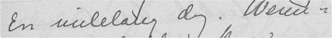En milelang dag. Weren-
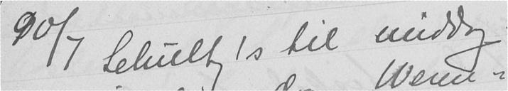10/7 Schultz's til middag.
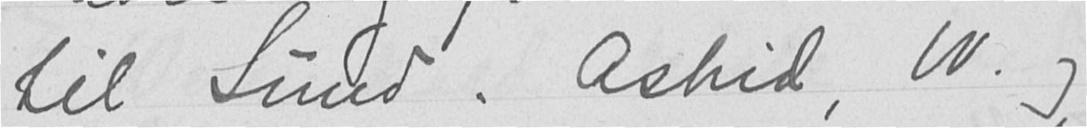til Sund. Astrid, W. og
